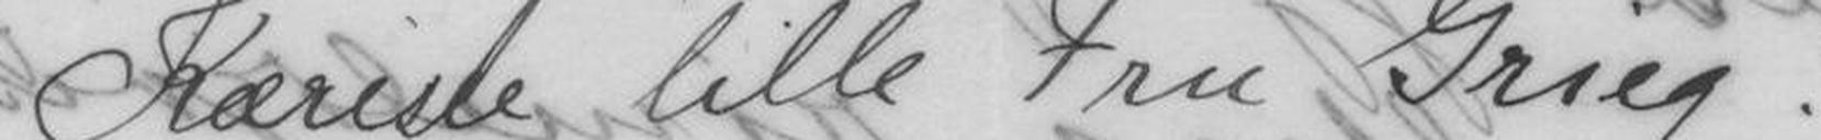Kæreste lille Fru Grieg!
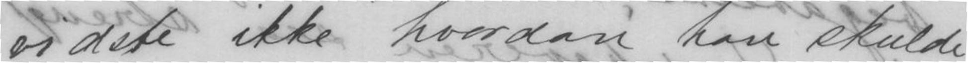vidste ikke hvordan han skulde
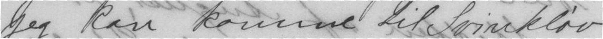jeg kan komme til Svinkløv
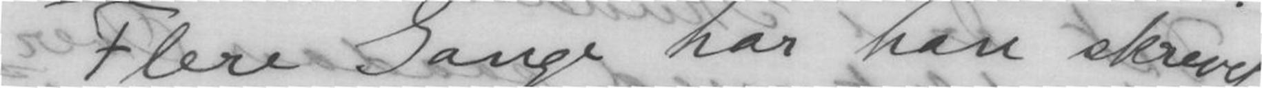Flere Gange har han skrevet
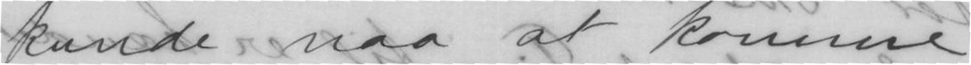kunde naa at komme
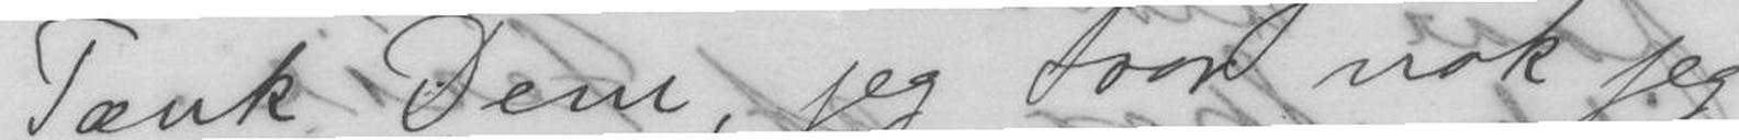Tænk Dem, jeg tror nok jeg
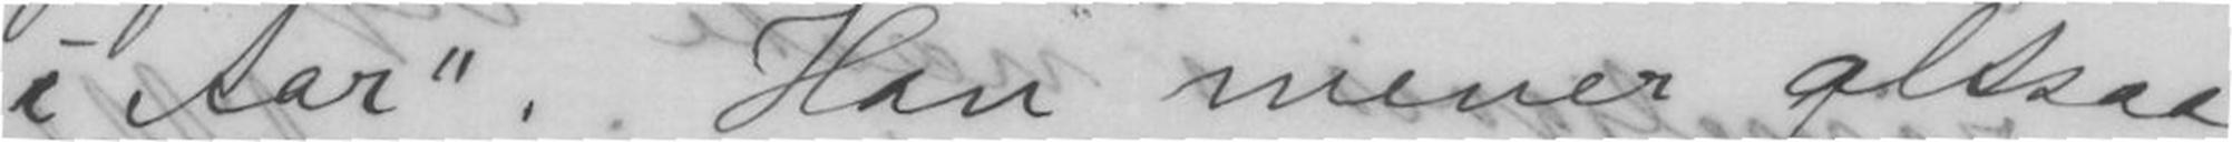i Aar. Han mener altsaa
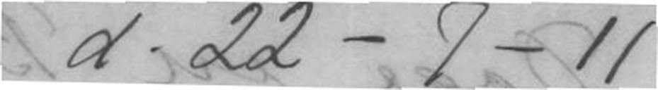d. 22- 7 -11
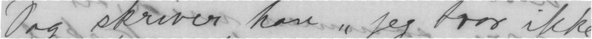Dag skriver han Jeg tror ikke
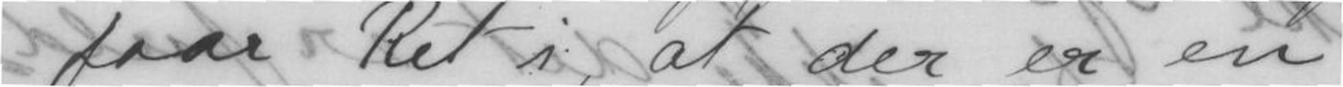faar Ret i at der er en
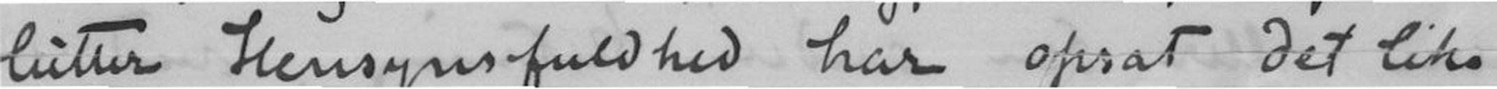lutter Hensynsfuldhed har opsat det like
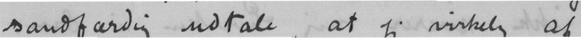sandfærdig udtale, at jeg virkelig af
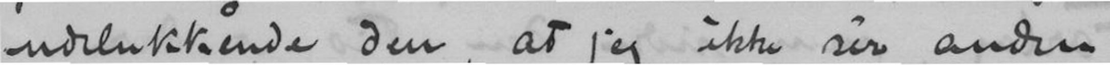udelukkende den, at jeg ikke sér anden
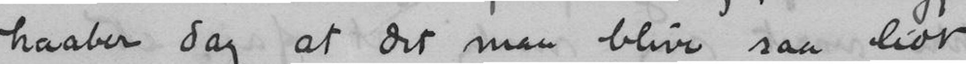haaber dog at det maa blive saa lidt
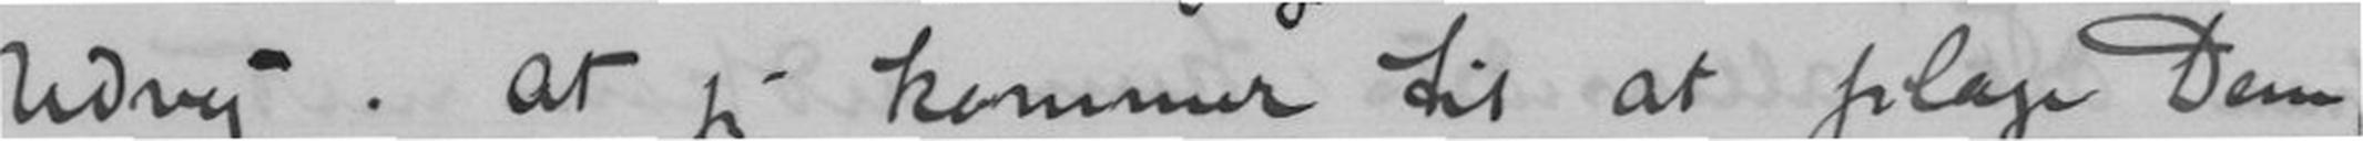Udvej. At jeg kommer til at plage Dem,
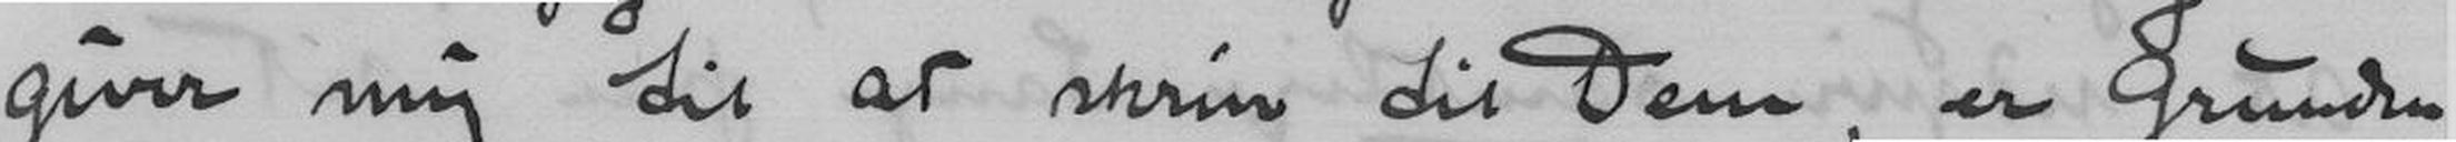giver mig til at skrive til Dem, er Grunden
